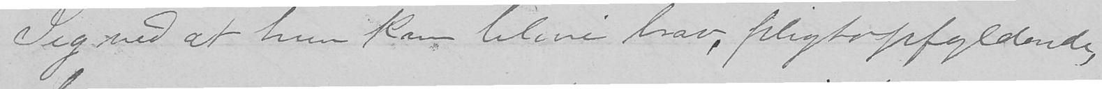Jeg ved at hun kan blive brav, pligtopfyldende,
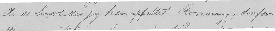de se hvorledes jeg har opfattet Rønnaug, derfor
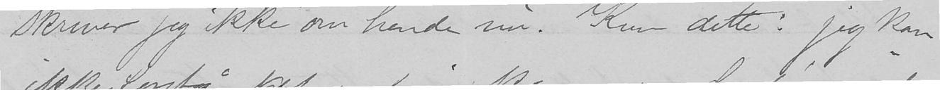skriver jeg ikke om hende nu. Kun dette: jeg kan
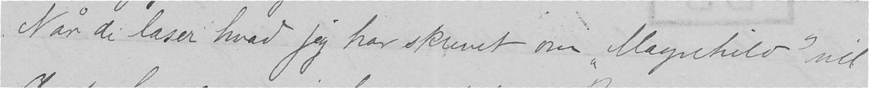Når de læser hvad jeg har skrevet om "Magnhild" vil
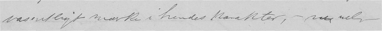væsentligt mærke i hendes karakter, - nu vel -
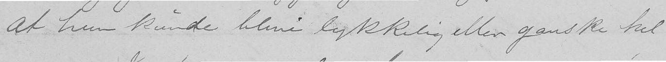at hun kunde blive lykkelig eller ganske hel
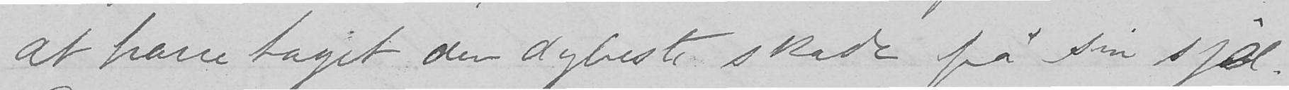at have taget den dybeste skade på sin sjæl.
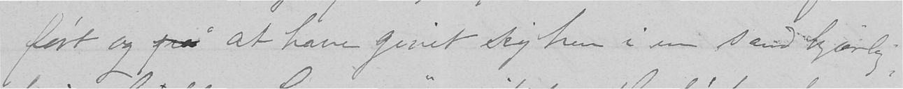ført og på at have givet sig hen i en sand kjærlig-
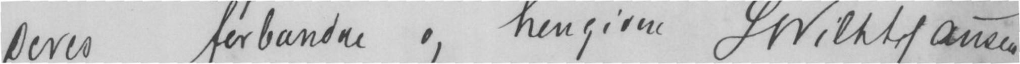Deres forbundne og hengivne Wilhelm Hansen
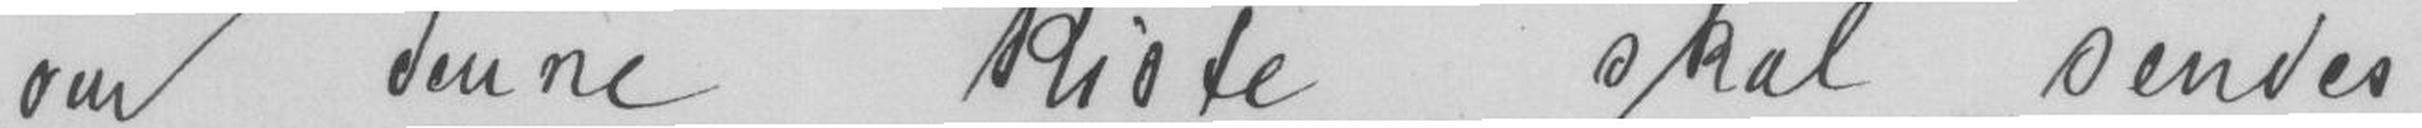om denne Kiste skal sendes
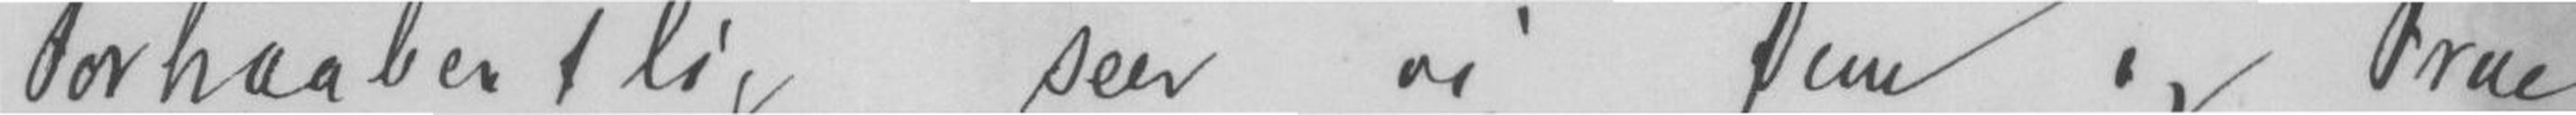Forhaabentlig ser vi Dem og Frue
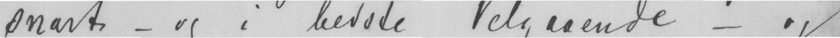snart - og i bedste Velgaaende - og
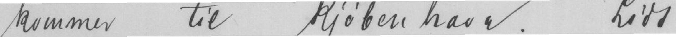kommer til Kjøbenhavn. Lidt
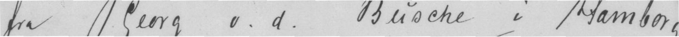fra Georg v. d. Busche i Hamborg
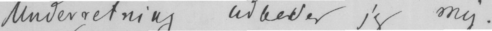underretning udbeder jeg mig.
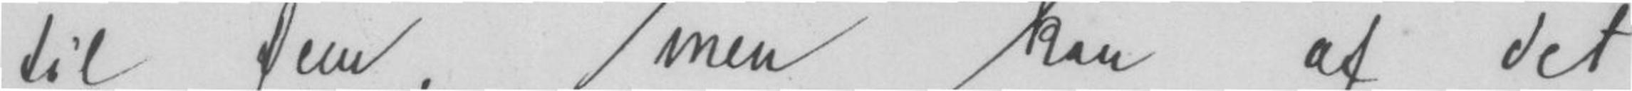til Dem, men kan af det
